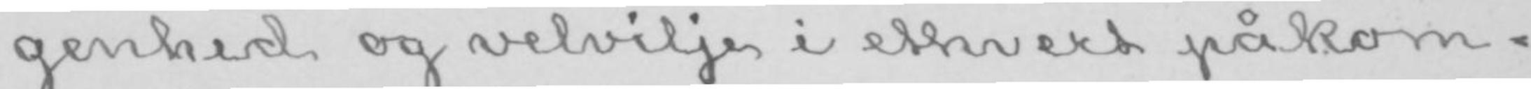genhed og velvilje i ethvert påkom-
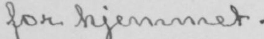for hjemmet.
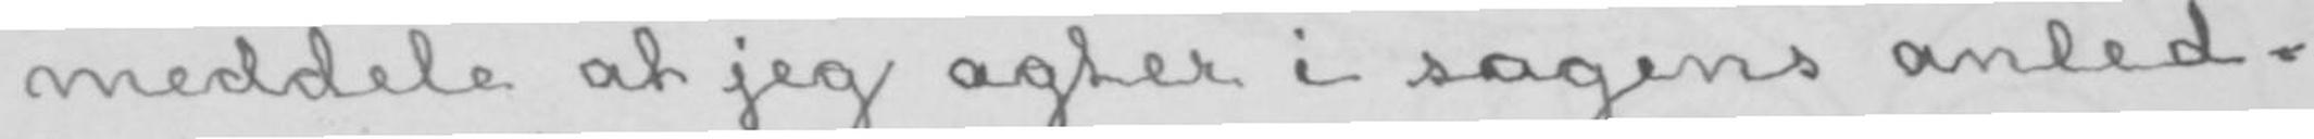meddele at jeg agter i sagens anled-
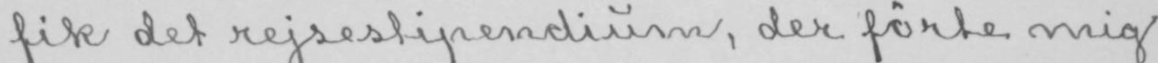fik det rejsestipendium, der førte mig
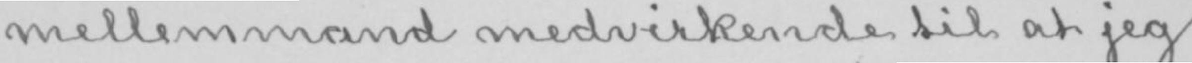mellemmand medvirkende til at jeg
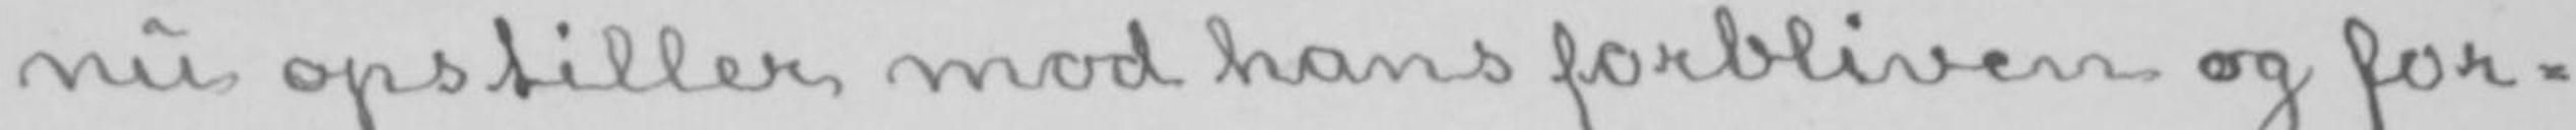nu opstiller mod hans forbliven og for-
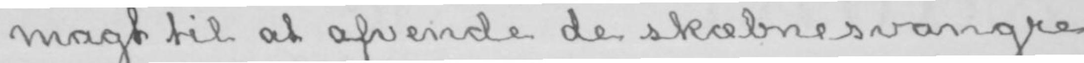magt til at afvende de skæbnesvangre
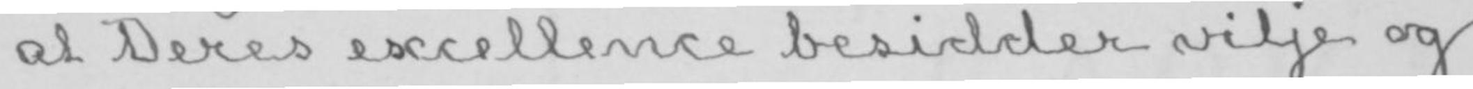at Deres excellence besidder vilje og
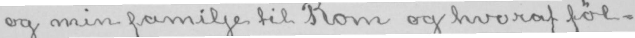og min familje til Rom og hvoraf føl-
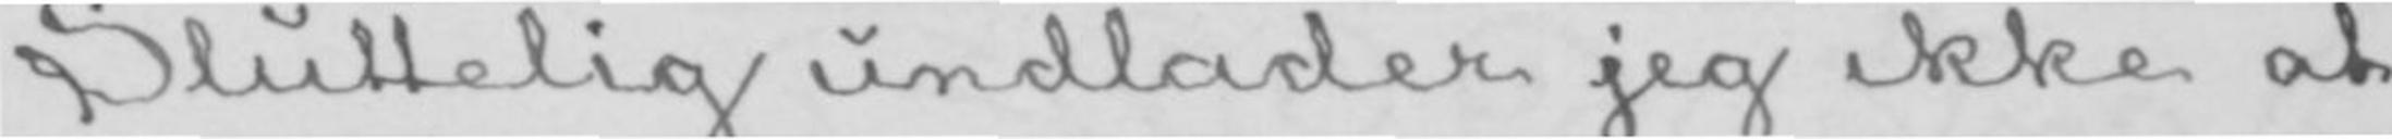Sluttelig undlader jeg ikke at
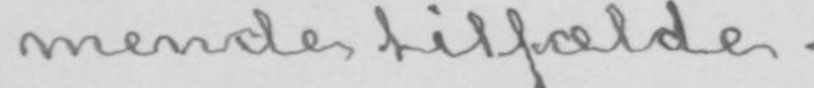mende tilfælde.-
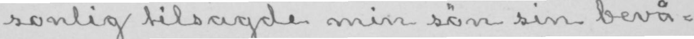sonlig tilsagde min søn sin bevå-
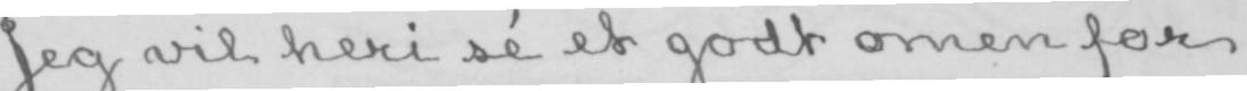Jeg vil heri sé et godt omen for
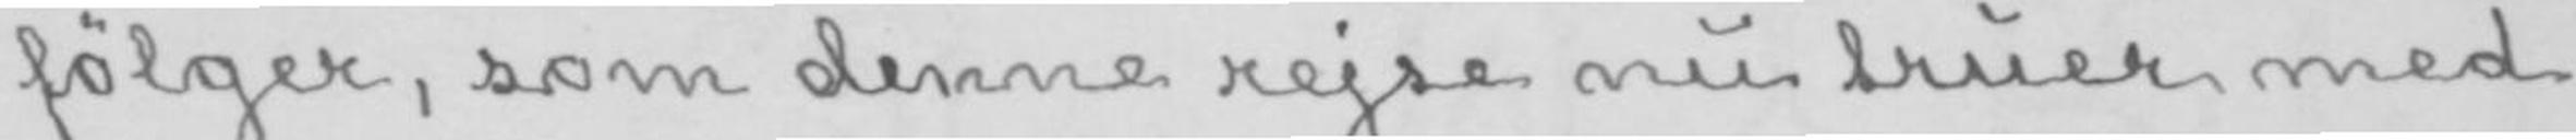følger, som denne rejse nu truer med
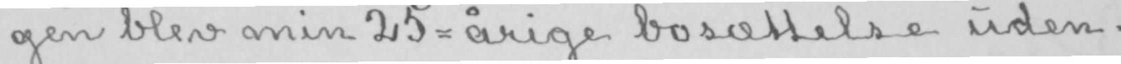gen blev min 25-årige bosættelse uden-
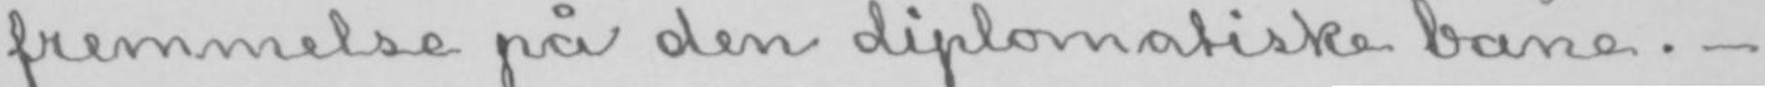fremmelse på den diplomatiske bane.-
